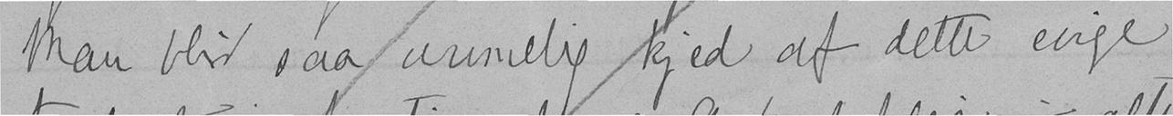Man blir saa urimelig kjed af dette evige
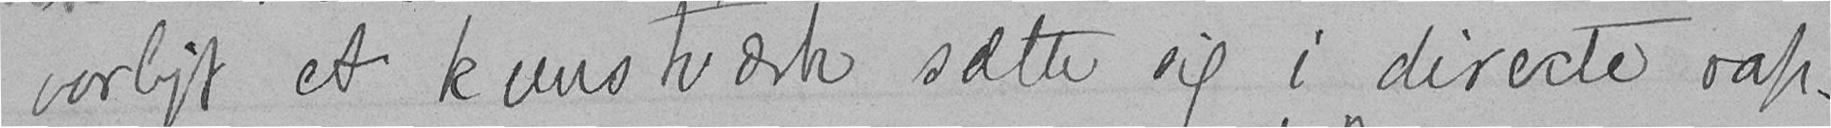vorligt et kunstværk sætte sig i directe rap-
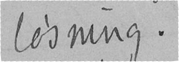løsning.
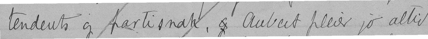tendents og partisnak, og Aubert pleier jo altid
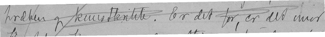præken og kunstkritik. Er det for, er det imod
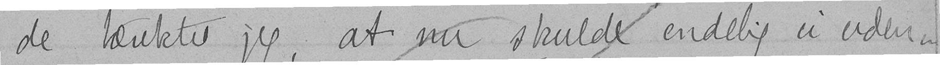de tænkte jeg, at nu skulde endelig vi uden-
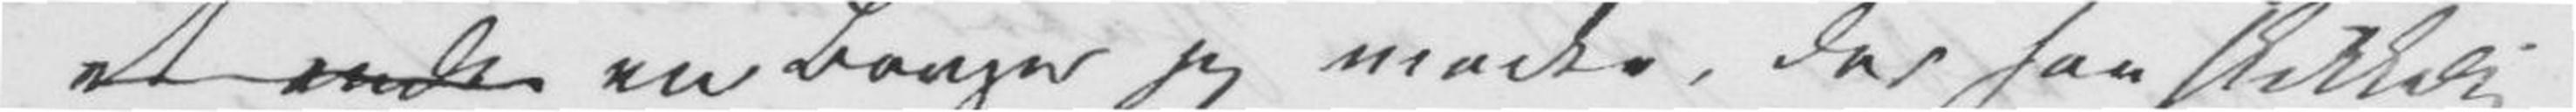et <gap /></del> en Langer jeg mødte, der saa skikkelig
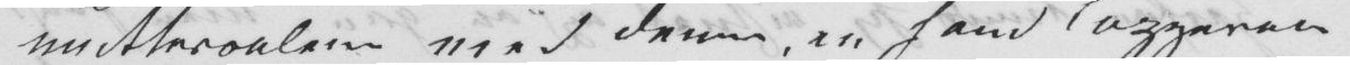muttersalene med denne, en sand Lazzaron
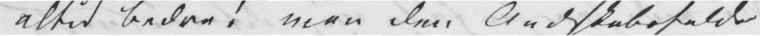altid bedre! men den Ondskabsfulde
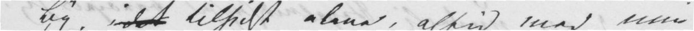By, idet tilsidst alene, altid med min
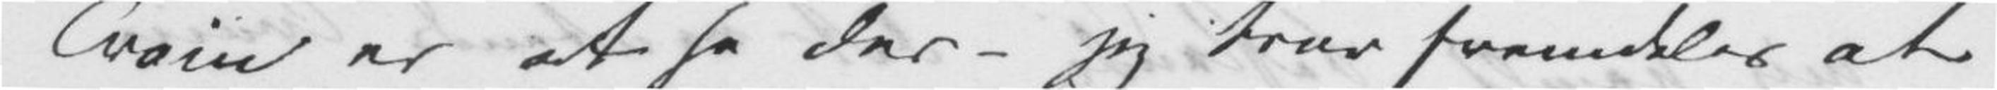Train er at se der – jeg tror fremdeles at
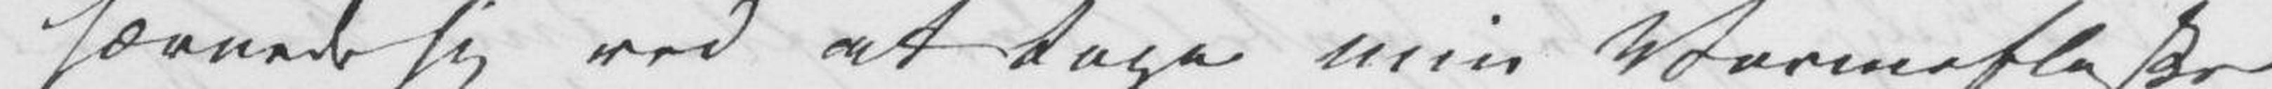hævnede sig ved at tage min Varmeflaske
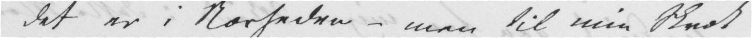det er i Nærheden – men til min Skræk
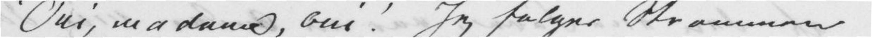«Oui, madame, oui![»] Jeg følger Strømmen
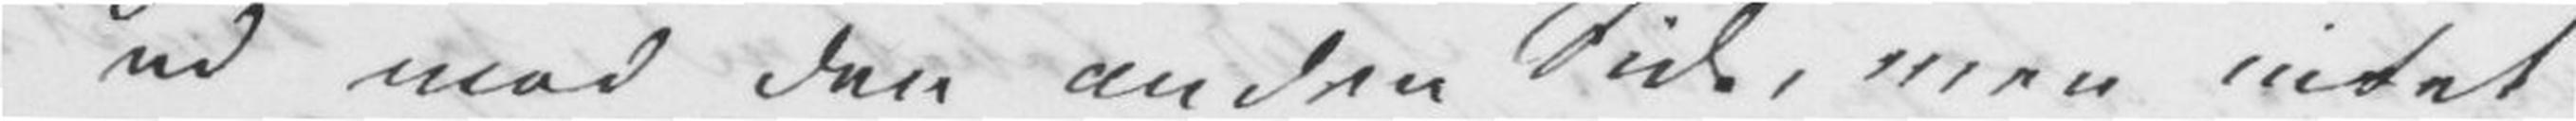ud mod den anden Side, men intet
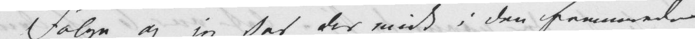Følge og jeg står der midt i den fremmede
